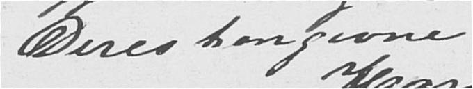Deres hengivne
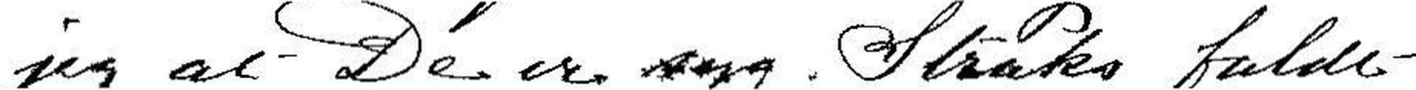jeg at De er syg. Straks faldt
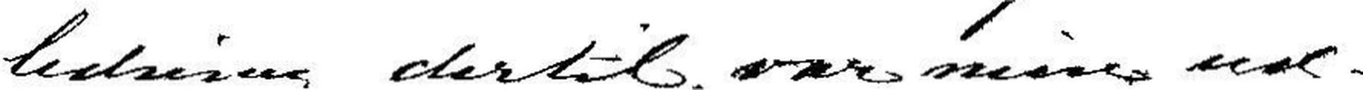ledning dertil, var mine ud-
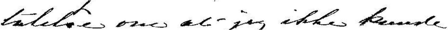talelse om at jeg ikke kunde
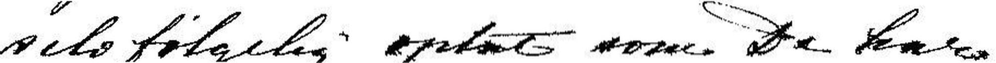selvfølgelig optat som De har
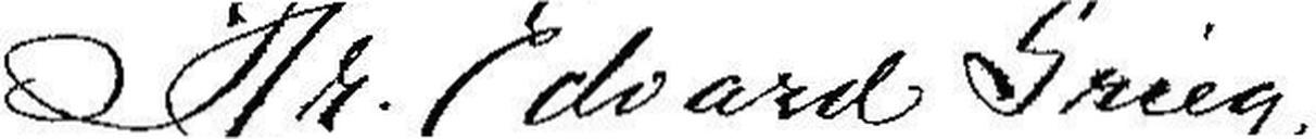Hr. Edvard Grieg
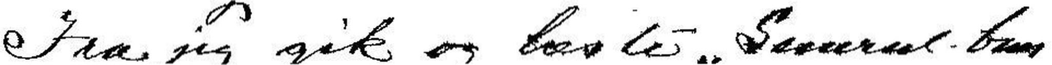Fra jeg gik og læste Semrul bus
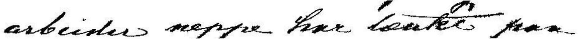arbeider neppe har tænkt paa
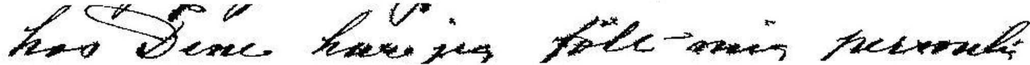hos Dine har jeg følt mig personlig
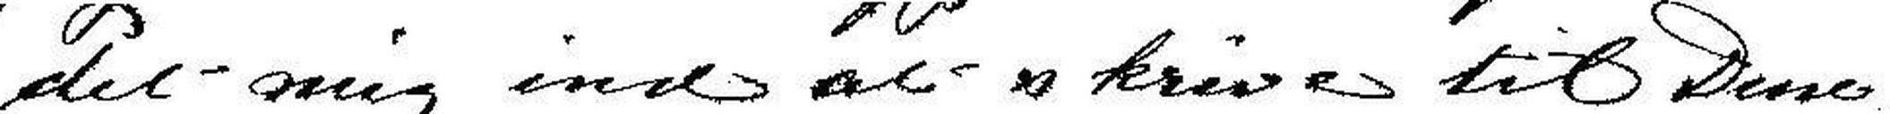det mig ind at skrive til Dem.
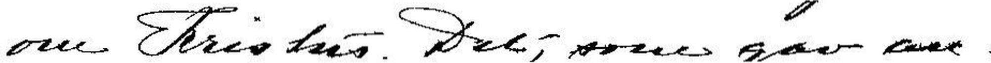om Kristus Det, som gav an-
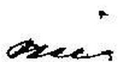mig.
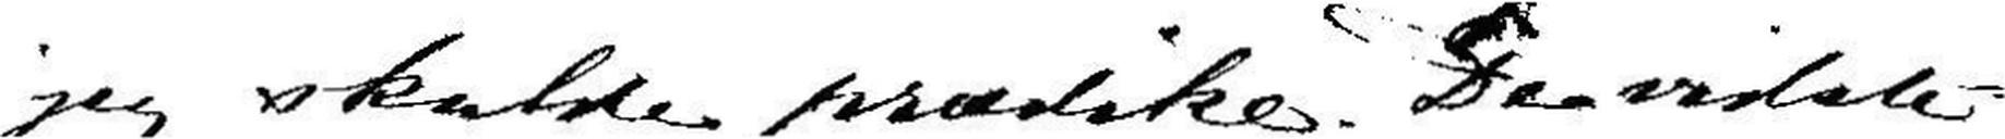jeg skulde prædike. De vidste
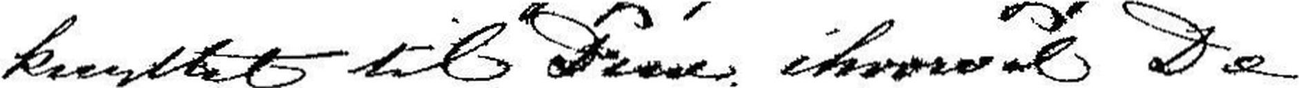knyttet til Dem, ihvorvel De
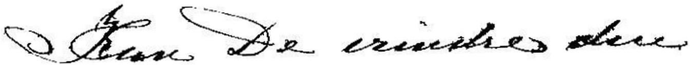Kan De erindre den
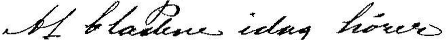Af bladerne idag hører
